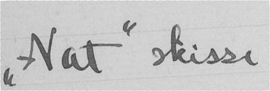"Nat" skisse
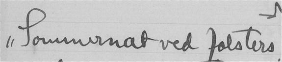"Sommernat ved Jølsters
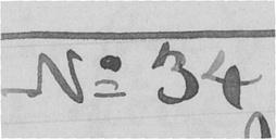No 34
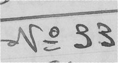No 33
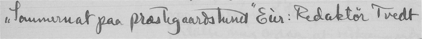"Sommernat paa præstegaardstunet" Eier: Redaktør Tvedt
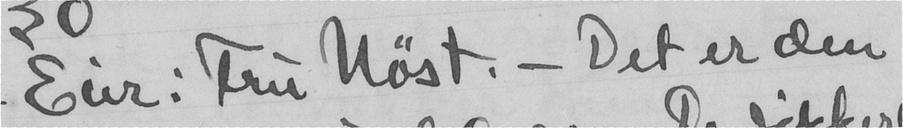Eier: Fru Høst. - Det er den
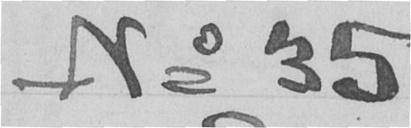No 35
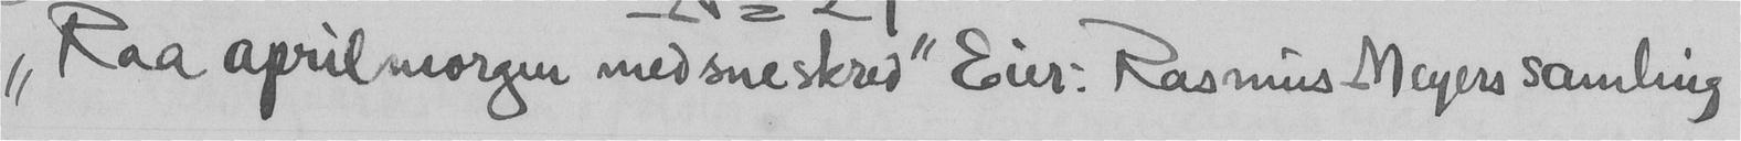"Raa aprilmorgen med sneskred" Eier. Rasmus Meyers samling
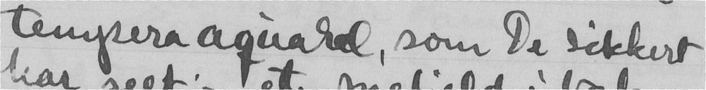temperaaquarel, som De sikkert
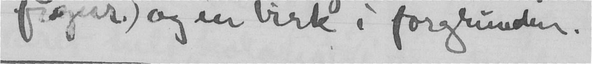figur) og en birk i forgrunden.
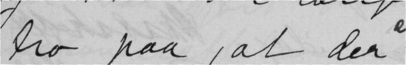tro paa, at der
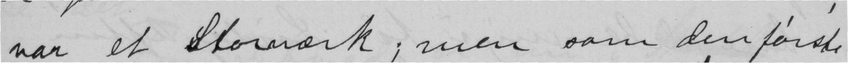var et Storværk; men som den første
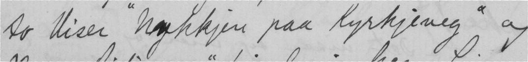to Viser "Nykkjen paa kyrkjeveg" og
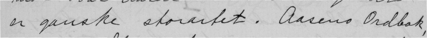er ganske storartet. Aasens Ordbok,
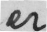er
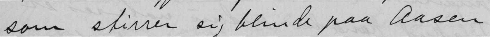som stirrer sig blinde paa Aasen
